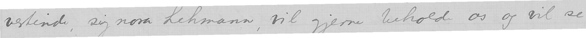vertinde, signora Lehmann, vil gjerne beholde os og vil se
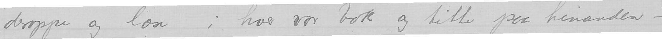deroppe og læse i hver vor bok og titte paa hinanden-
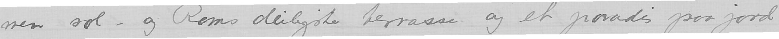men sol - og Roms deiligste terrasse og et paradis paa jord
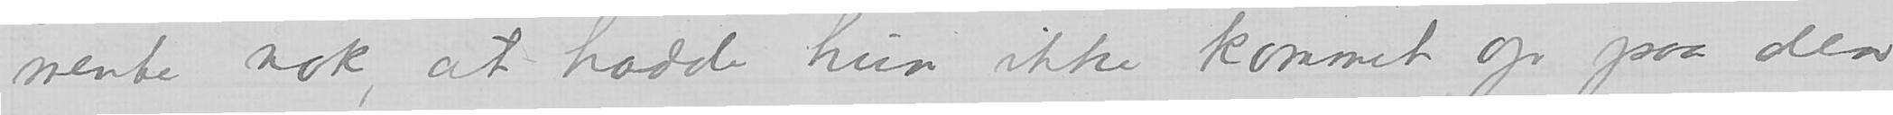mente nok, at hadde hun ikke kommet op paa den
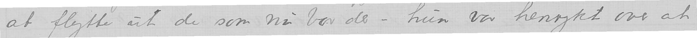at flytte ut de som nu bor der. hun var henrykt over at
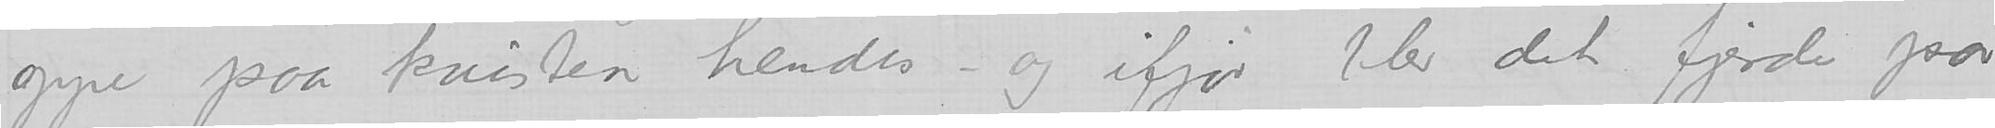oppe paa kvisten hendes – og ifjor blev det fjerde
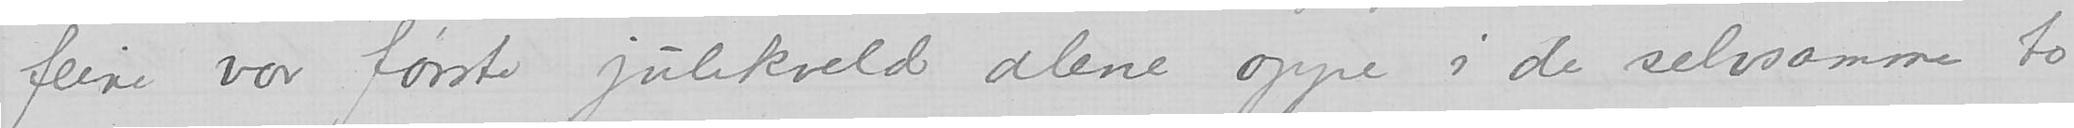feire vor første julekveld alene oppe i de selvsamme to
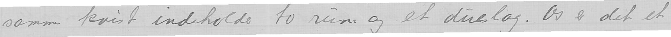samme kvist indeholder to rum og et dueslag. Os er det
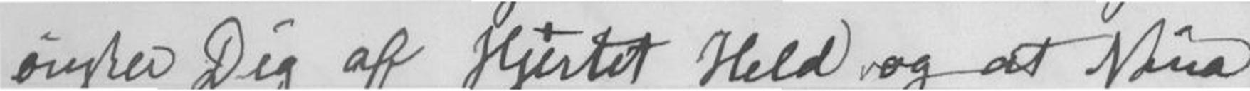ønsker Dig af Hjertet Held og at Nina
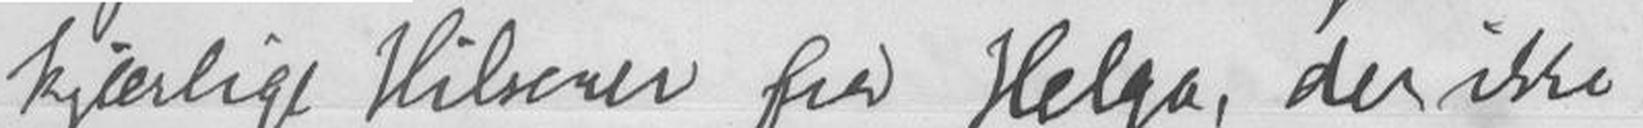kjærlige Hilsener fra Helga, der ikke
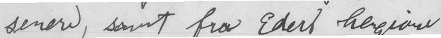senere, semt fra Eders hengivne
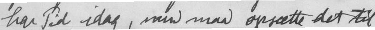har Tid idag, men maa opsætte det til
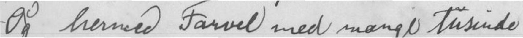Og hermed Farvel med mange tusinde
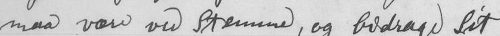maa være ved Stemme, og bidrage Sit
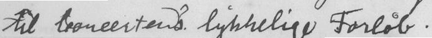til Concertens lykkelige Forløb.
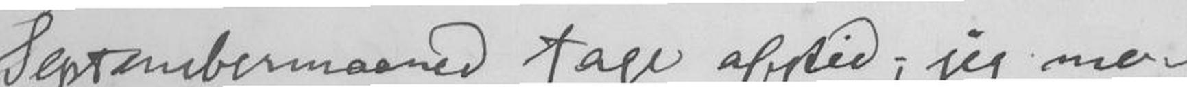Septembermaaned tage afsted; jeg me-
